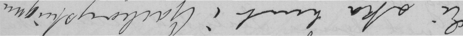Ei ska hent i Garborgstuguu
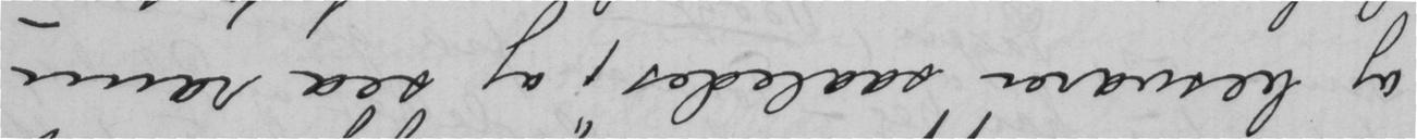og besvarer saaledes," og sea rann
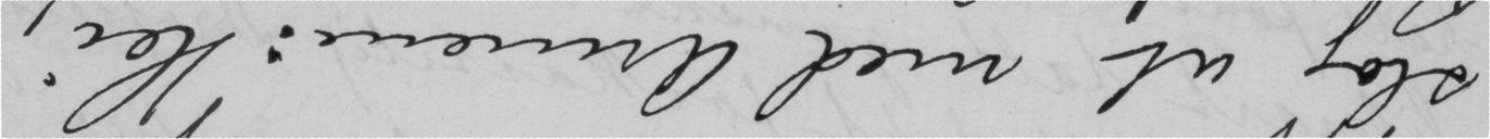slog ut med armene: Hei,
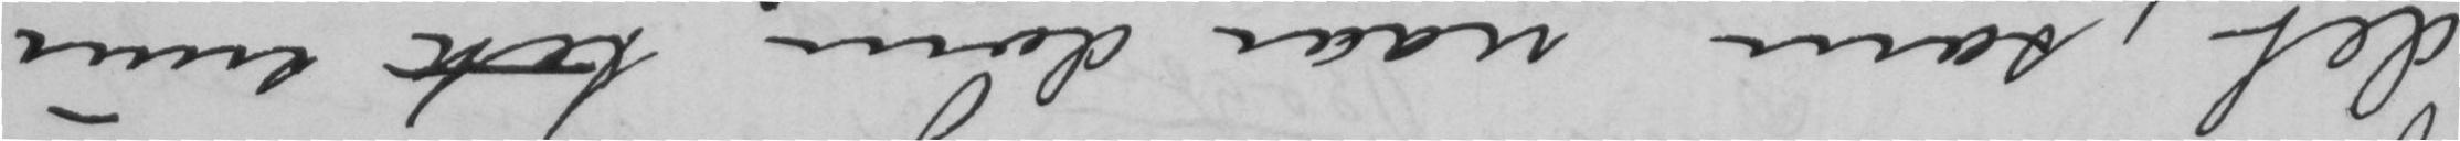det, som naa dom  snur
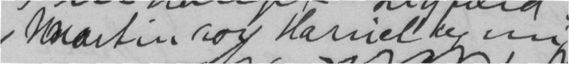Martin roy Harriet ky nu;
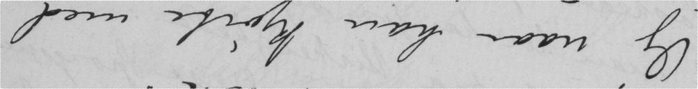Og naar han kjørte med
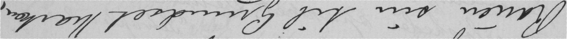Rauen sin til Grundset Marken,
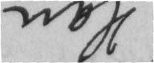Han
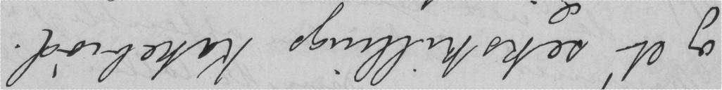og et sekskillings Kakebrød.
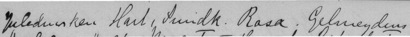Juledunken Hart, Smidk. Rosa. Gelmeydens
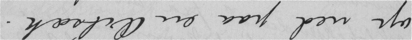op ned paa en Ærtsæk.
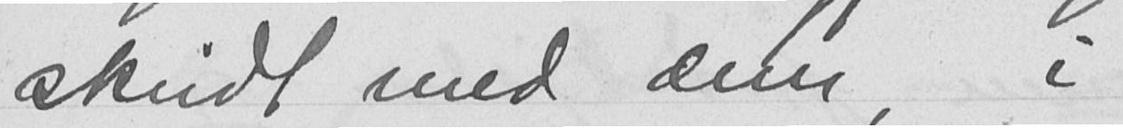skridt med dem, i
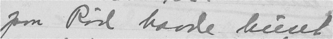paa Rød havde huset
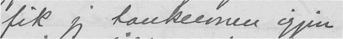fik jeg tankeevnen igjen
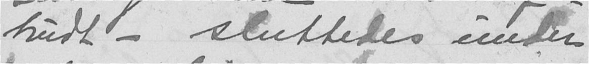brudt - sluttedes under
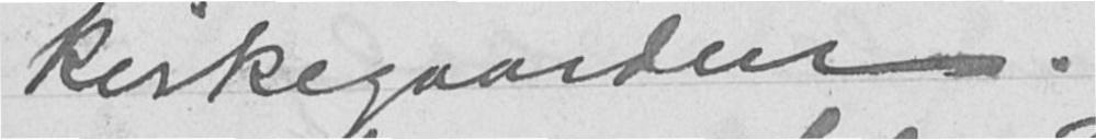kirkegaarden.
